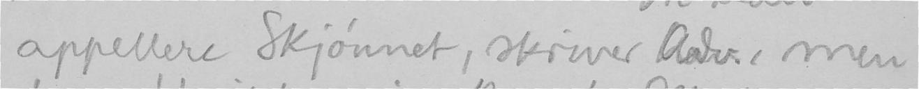appellere Skjønnet, skriver Adv., men
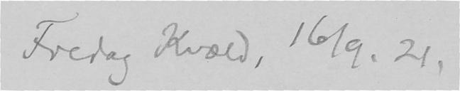Fredag Kvæld, 16/9. 21.
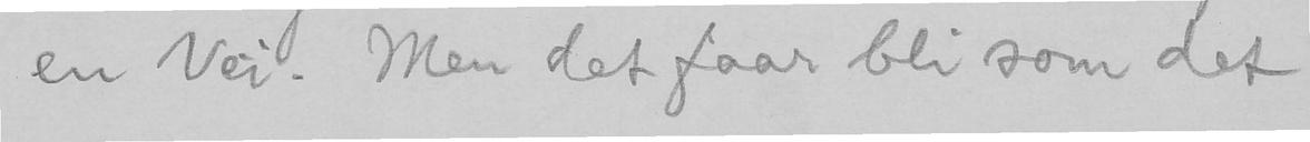en Vei. Men det faar bli som det
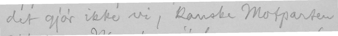det gjør ikke vi, kanske Motparten
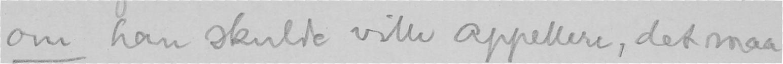om han skulde ville appellere, det maa
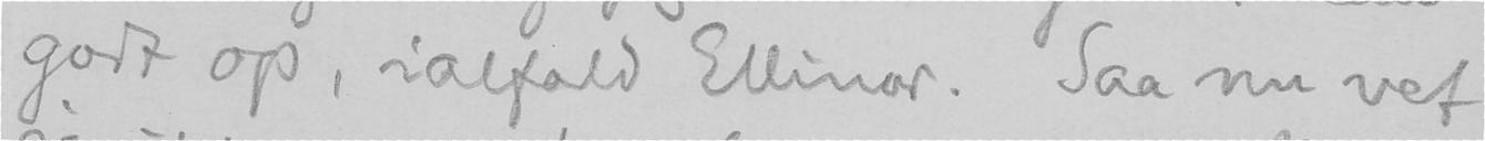godt op, ialfald Ellinor. Saa nu vet
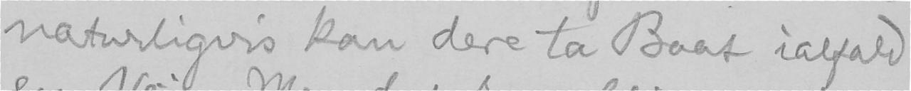naturligvis kan dere ta Baat ialfald
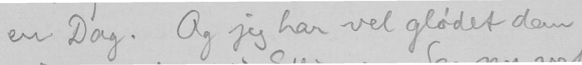en Dag. Og jeg har vel glødet dem
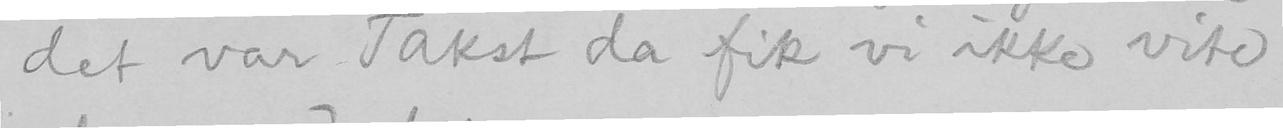det var Takst da fik vi ikke vite
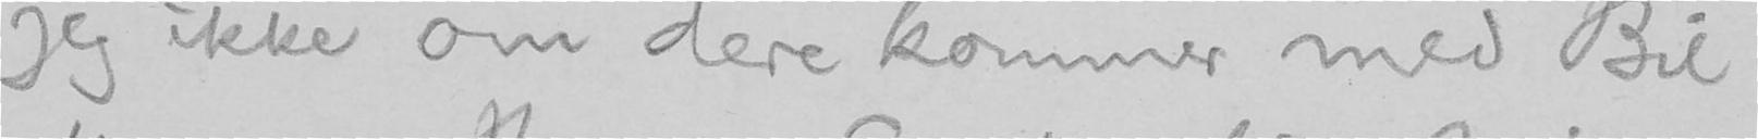jeg ikke om dere kommer med Bil
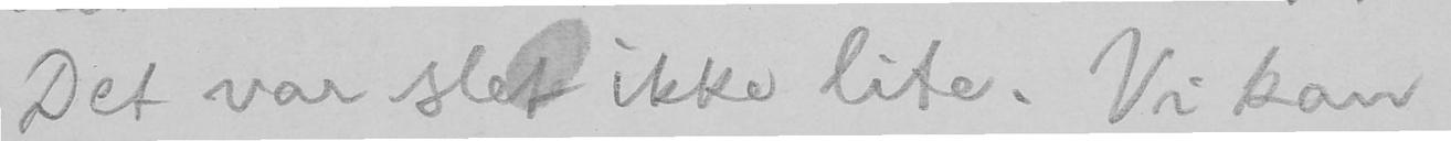Det var slet ikke lite. Vi kan
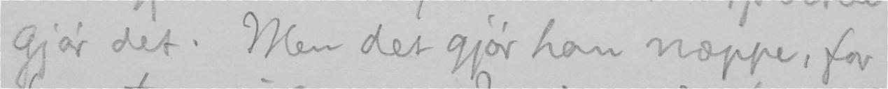gjør det. Men det gjør han næppe, for
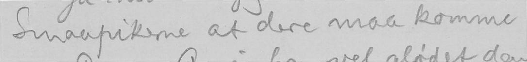Smaapikerne at dere maa komme
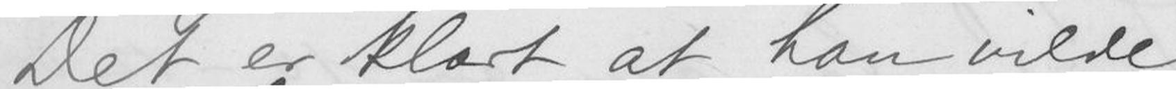Det er klart at han vilde
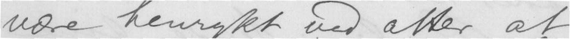være henrykt ved atter at
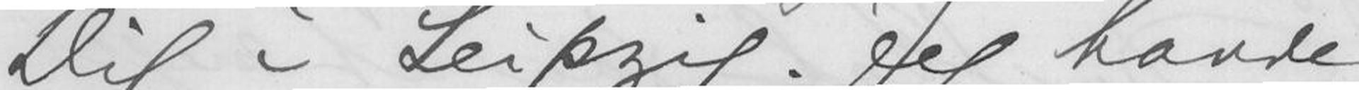Dig i Leipzig. Jeg havde
